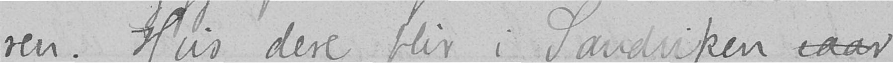ren. Hvis dere blir i Sandviken iaar
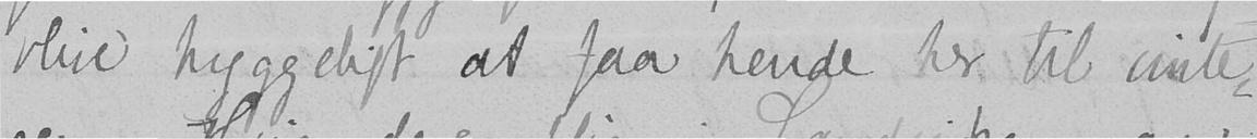blive hyggeligt at faa hende her til vinte-
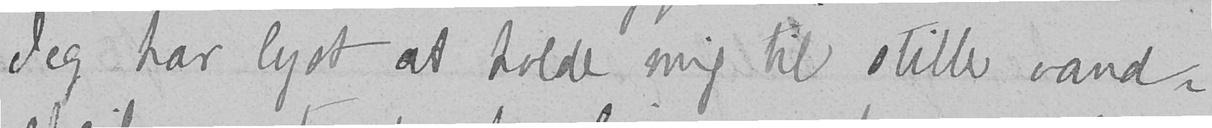Jeg har lyst at holde mig til stille vand-
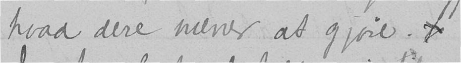hvad dere mener at gjøre. X
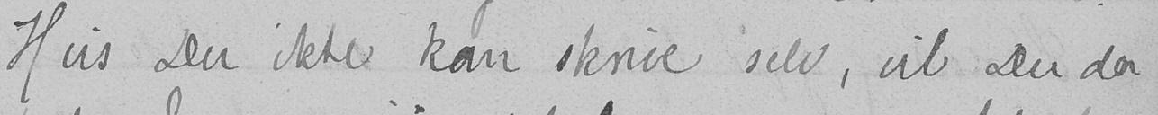Hvis Du ikke kan skrive selv, vil Du da
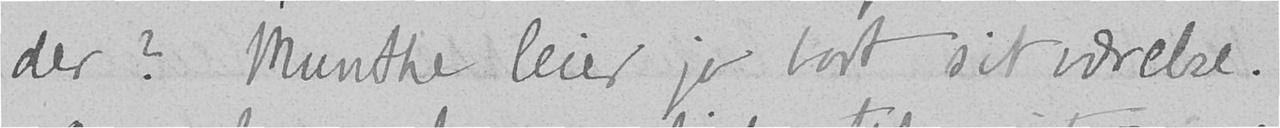der? Munthe leier jo bort sit værelse.
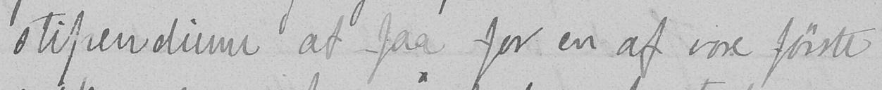stipendium at faa for en af vore første
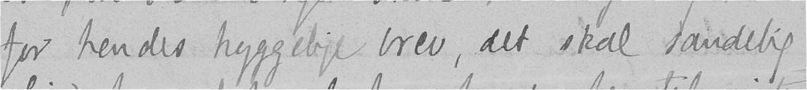for hendes hyggelige brev, det skal sandelig
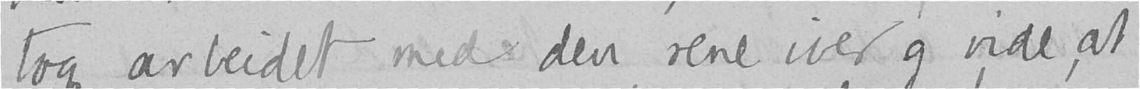tog arbeidet med den rene iver og vide, at
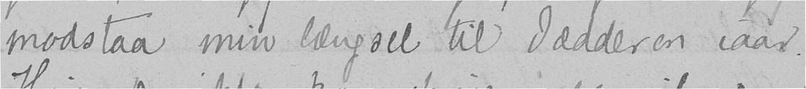modstaa min længsel til Jædderen iaar.
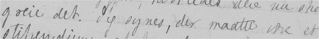greie det. Jeg synes, der maatte være et
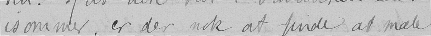isommer, er der nok at finde at male
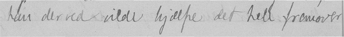han derved vilde hjælpe det hele fremover
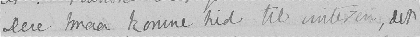Dere maa komme hid til vinteren, det
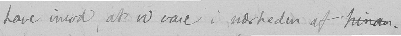have imod, at vi vare i nærheden af hinan-
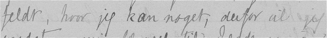feldt, hvor jeg kan noget, derfor vil jeg
